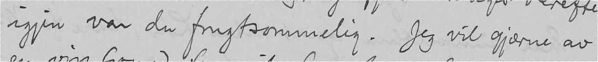igjen var du frugtsommelig. Jeg vil gjærne av
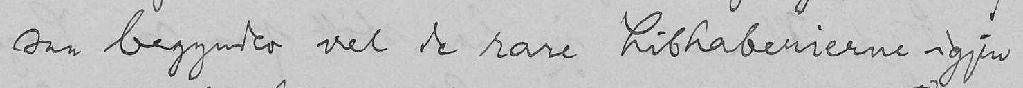saa begynder vel de rare Libhaberierne igjen
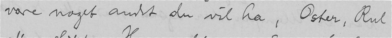være noget andet du vil ha, Oster, Rul
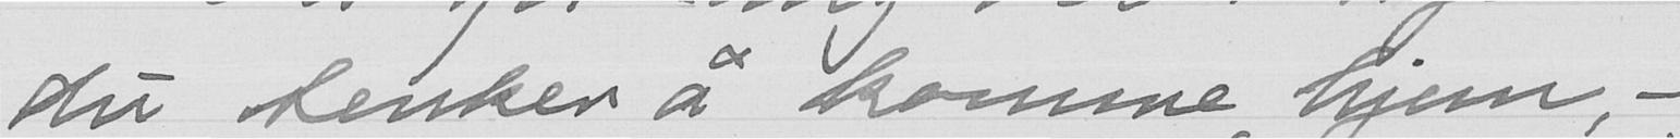du tenker å komme hjem -
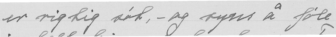er rigtig sat,- og syns å føle
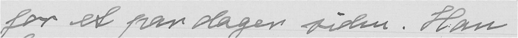for et par dager siden. Han
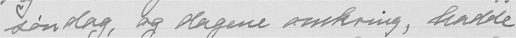søndag, og dagene omkring, hadde
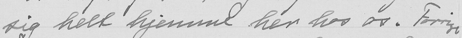sig helt hjemme her hos os. Forrige
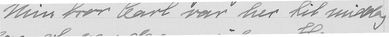Min bror Karl var her til middag
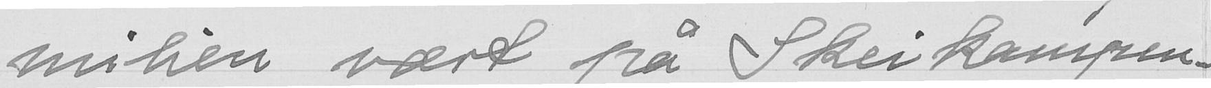milien været på Skeikampen-
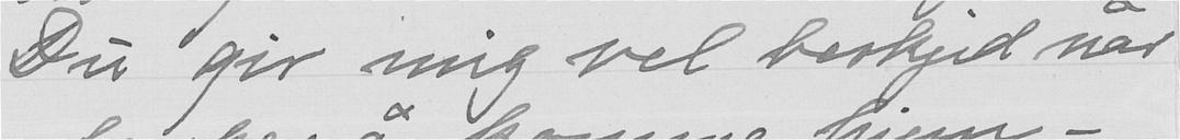Du gir mig vel beskjed når
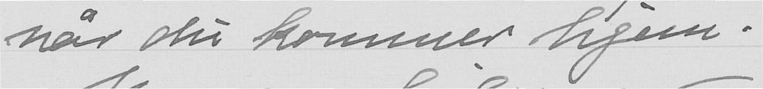når du kommer hjem!
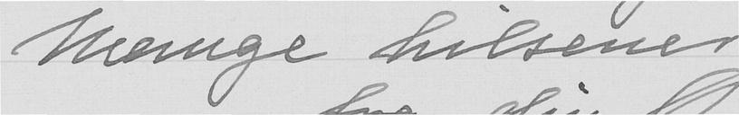Mange hilsener
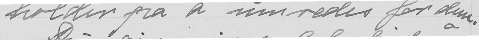holder på å innredes for dem.
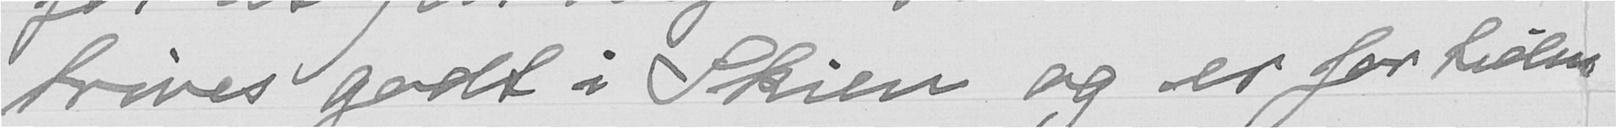trives godt i Skien og er for tiden
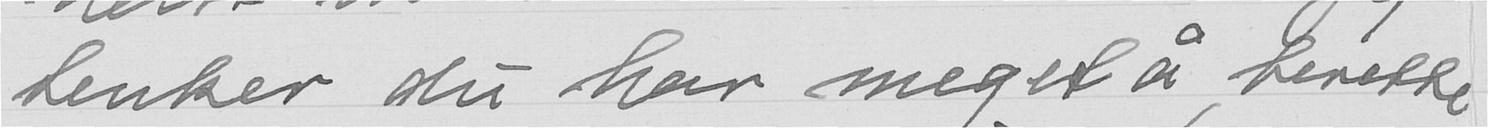tenker du har meget å berette
